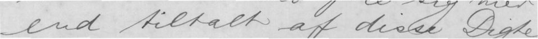end tiltalt af disse Digte,
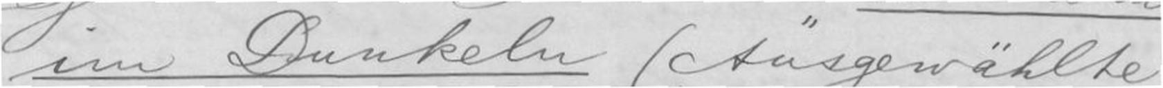im Dunkeln (Ausgewählte
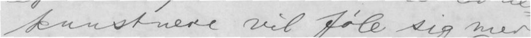kunstnere vil føle sig mer
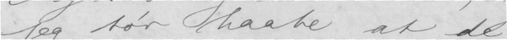jeg tør haabe at de
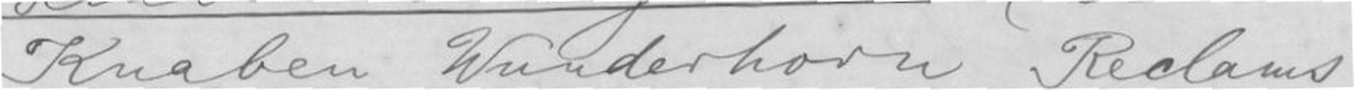Knaben Wunderhorn, Reclams
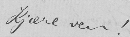Kjære ven!
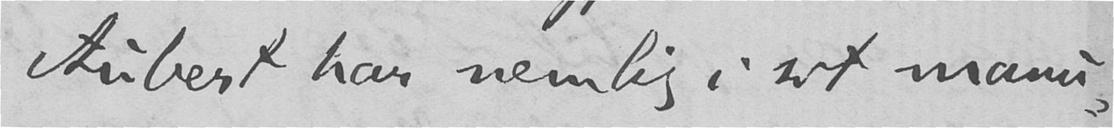Aubert har nemlig i sit manu-
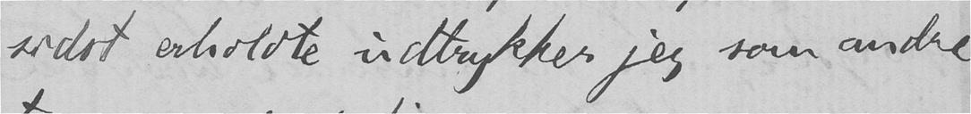sidst erholdte udtrykker jeg som andre
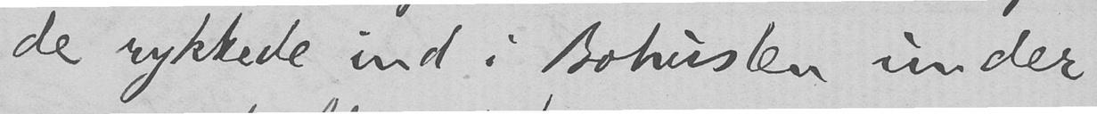de rykkede ind i Bohuslen under
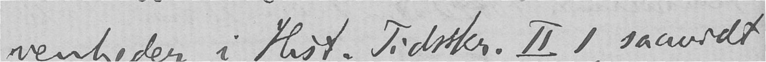venheder i Hist. Tidsskr. II 1, saavidt
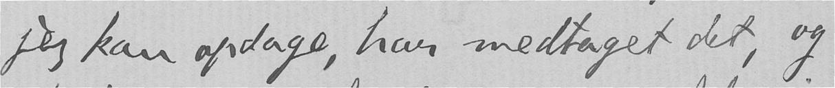jeg kan opdage, har medtaget det, og
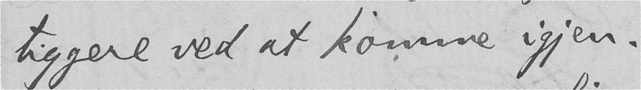tiggere ved at komme igjen.
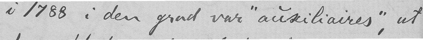i 1788 i den grad var "auxiliaires", at
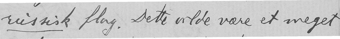russisk flag. Dette vilde være et meget
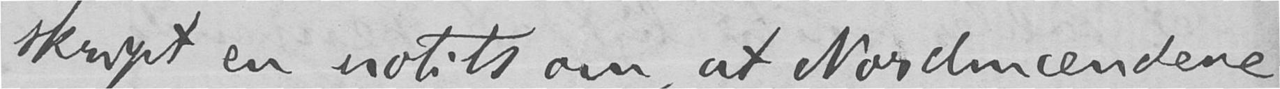skript en notits om, at Nordmændene
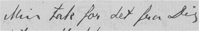Min tak for det fra Dig
